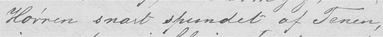Hørren snart spundet af Tenen,
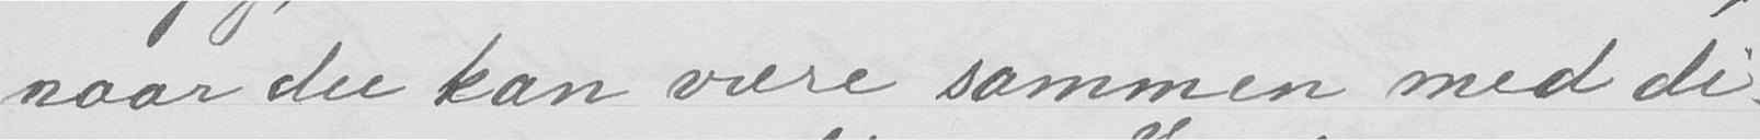naar du kan være sammen med di-
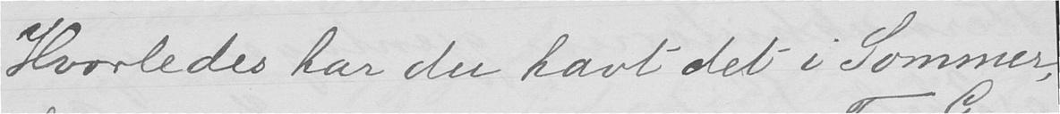Hvorledes har du havt det i Sommer,
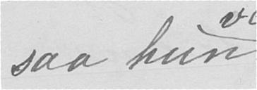saa hun
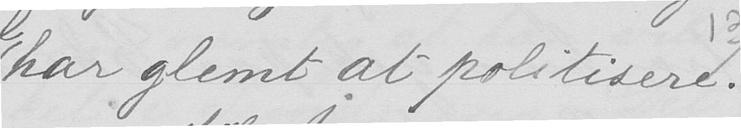har glemt at politisere.
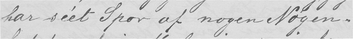har séet Spor af nogen Nøgen-
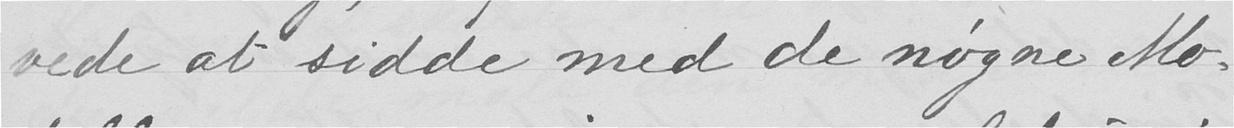vede at sidde med de nøgne Mo-
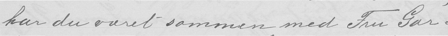har du været sammen med Fru Gar-
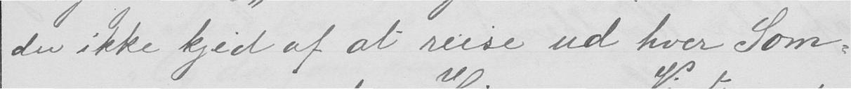du ikke kjed af at reise ud hver Som-
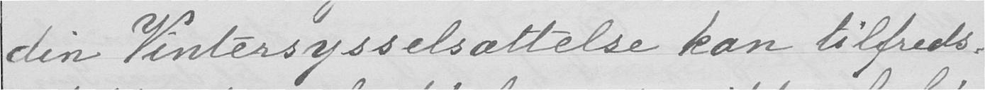din Vintersysselsættelse kan tilfreds-
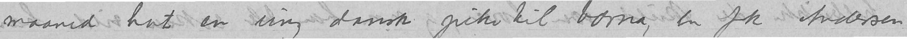maaned hat en ung danske pike til barna, en frk Andersen
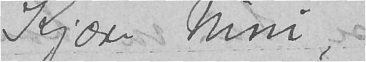Kjære Nini,
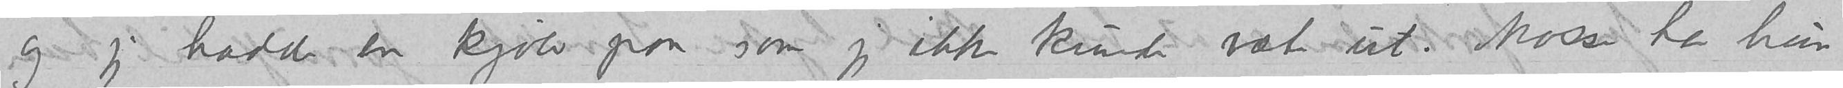og jeg hadde en kjole paa som jeg ikke kunde væte ut. Mosse har hun
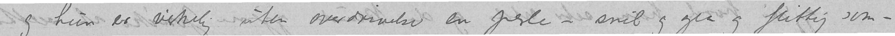og hun er virkelig uten overdrivelse en perle – snil og gla og flittig som –
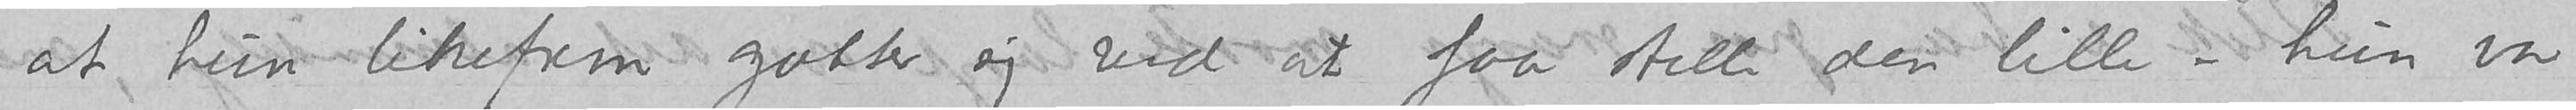at hun likefrem gotter sig ved at faa stelle den lille – hun var
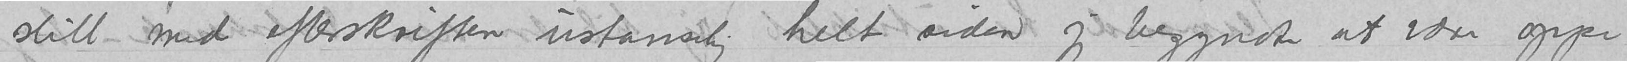slitt med efterskriften ustanselig helt siden jeg begyndte at være oppe
